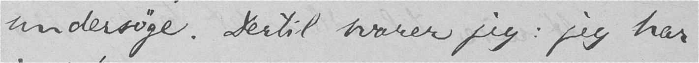undersøge. Dertil svarer jeg: jeg har
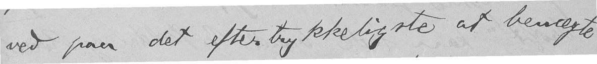ved paa det eftertrykkeligste at benægte
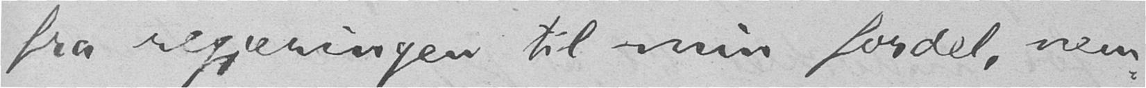fra regjeringen til min fordel, nem-
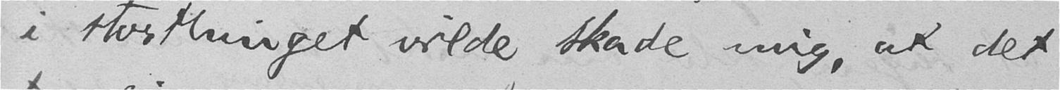i storthinget vilde skade mig, at det
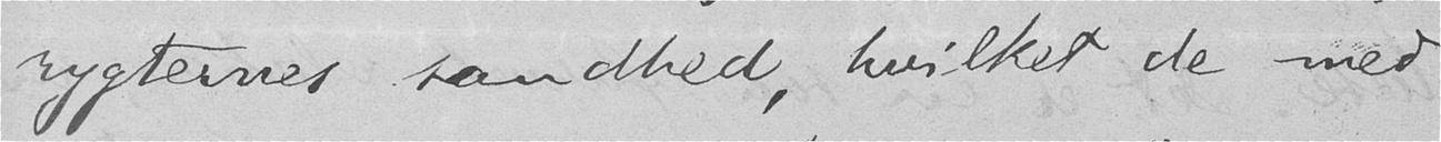rygternes sandhed, hvilket de med
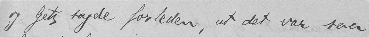og Getz sagde forleden, at det var saa
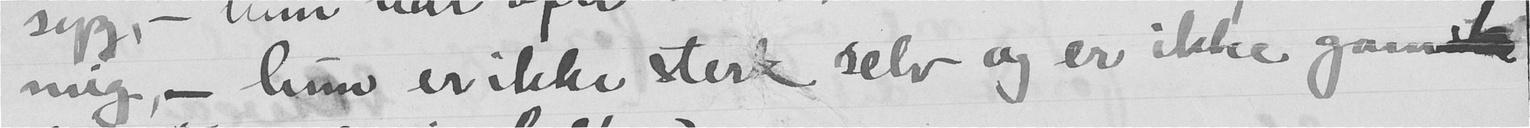mig, - hun er ikke sterk selv og er ikke ganske
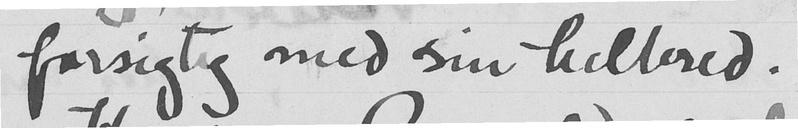forsigtig med sin helbred.
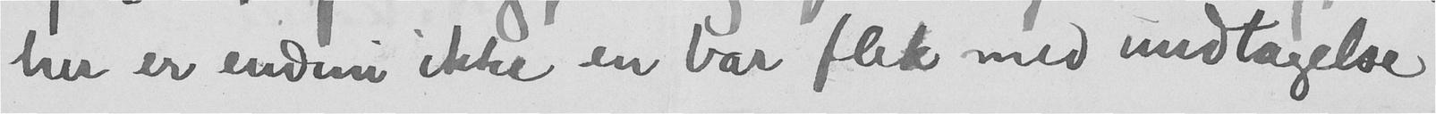her er endnu ikke en bar flek med undtagelse
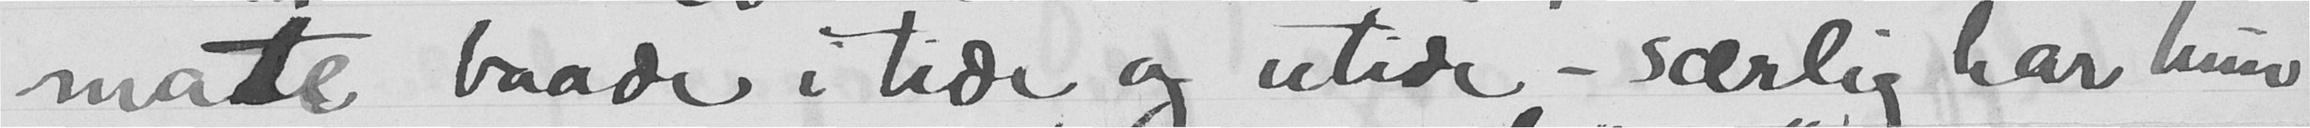mate baade i tide og utide - særlig har hun
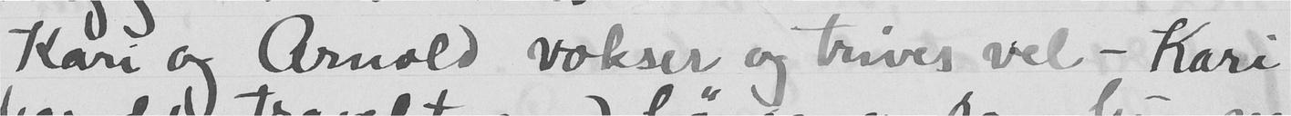Kari og Arnold vokser og trives vel - Kari
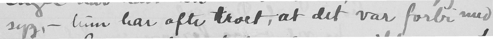syg, - hun har ofte troet, at det var forbi med
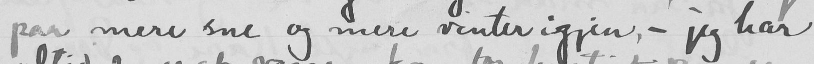par mere sne og mere vinter igjen, - jeg har
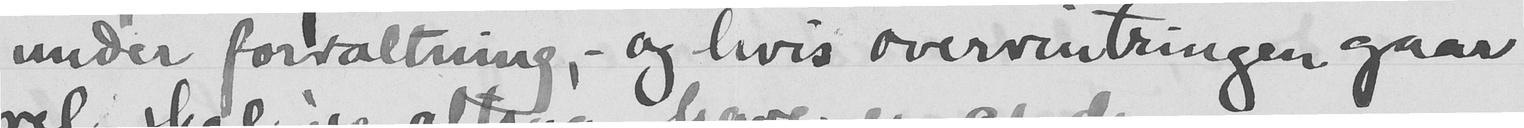under forvaltning, - og hvis overvintringen gaar
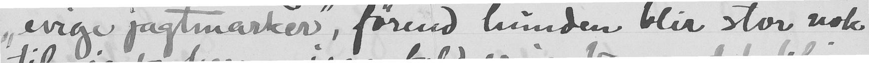"evige jagtmarker", førend hunden blir stor nok
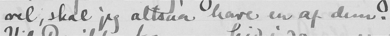vel, skal jeg altsaa have en af dem.
